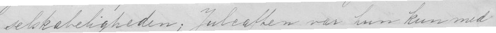selskabeligheden, Juleaften var hun kun med
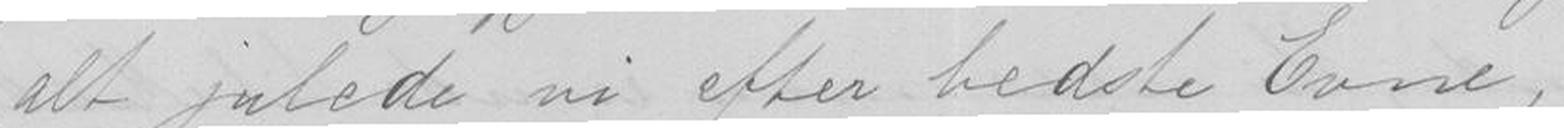alt julede vi efter bedste Evne, morso
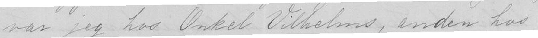var jeg hos Onkel Vilhelms, anden hos
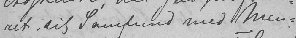ret til Samfund med Men-
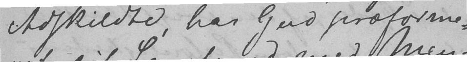Adskildte, har Gud præforme-
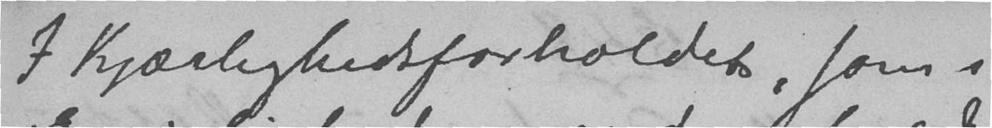I Kjærlighedsforholde, som i
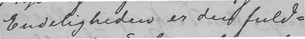Endeligheden er den fuld-
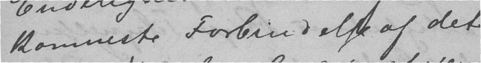komneste Forbindelse af det
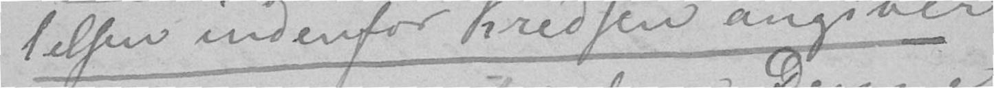lelsen indenfor Kredsen angiver
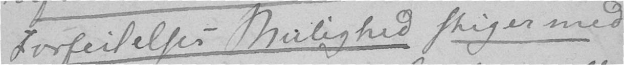Forfeilelses Mulighed stiger med
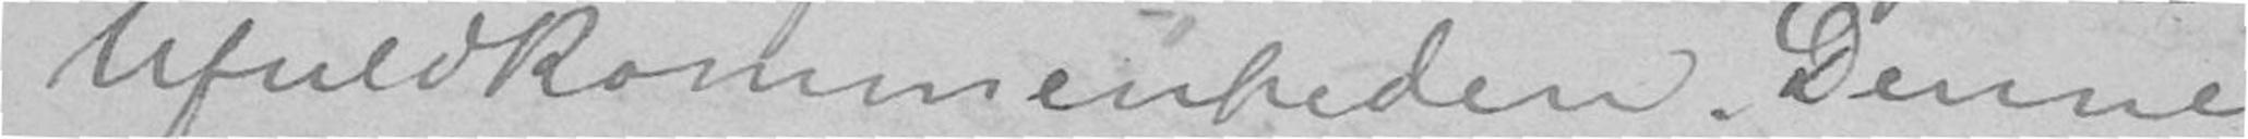Ufuldkommenheden. Denne
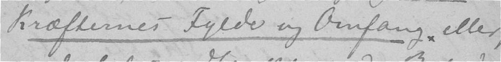Kræfternes Fylde og Omfang eller,
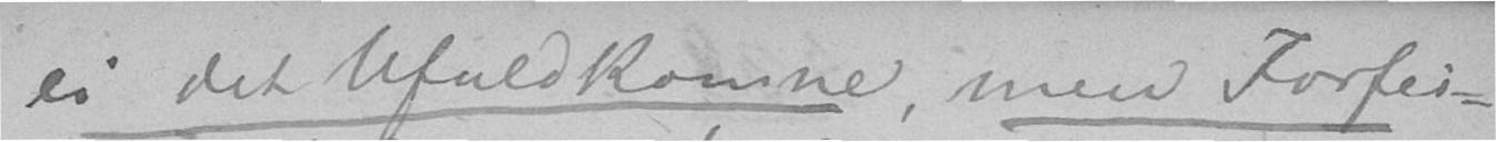er det Ufuldkomne, men Forfei-
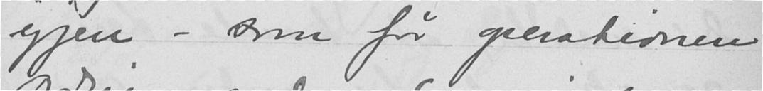igjen - som før operationen.
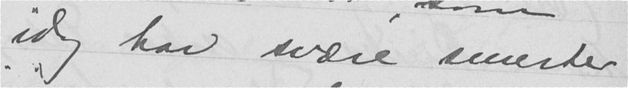idag har svære smerter
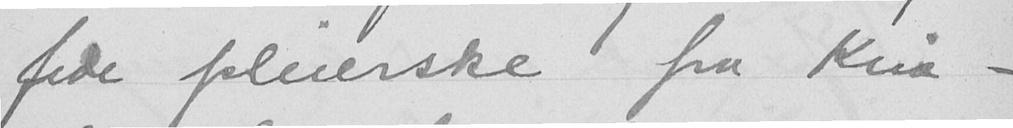fede pleierske fra Kria -
